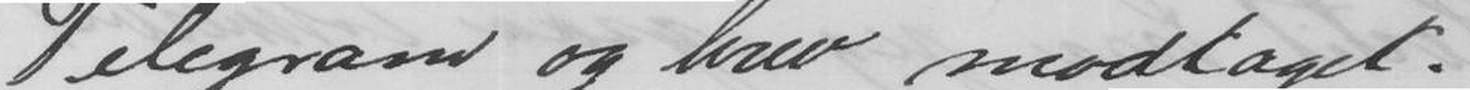Telegram og brev modtaget. -
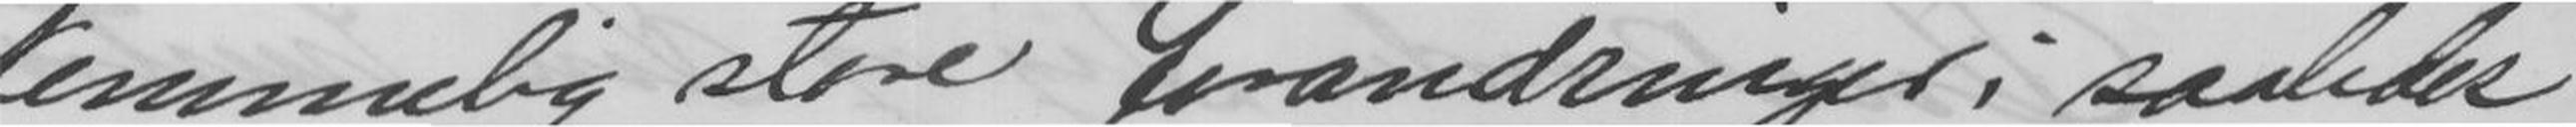temmelig store forandringer; saaledes
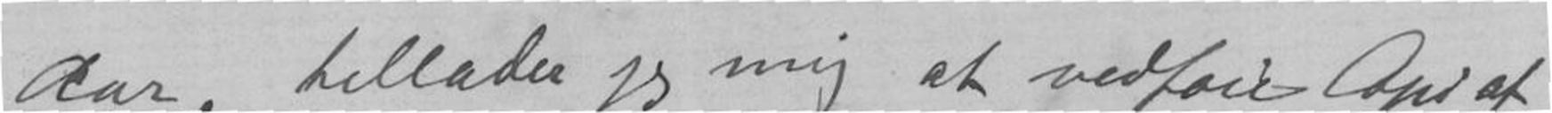Aar, tillader jeg mig at vedføie Ops af
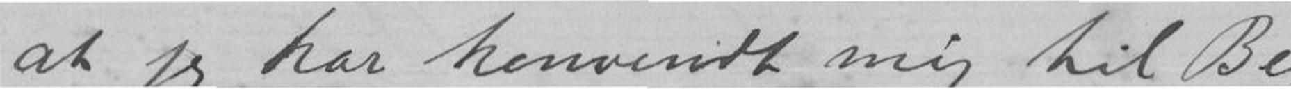at jeg har henvendt mig til Be
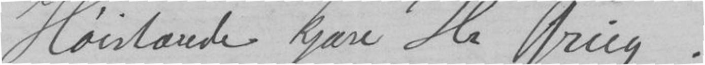Høistærde kjære Hr Grieg!
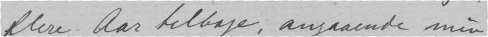flere Aar tillbage, angaaende min
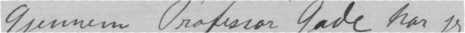Gjennem Professor Gade har jeg
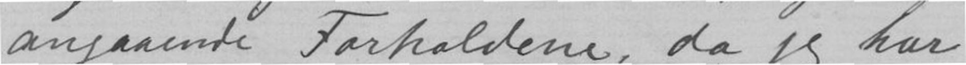angaaende Forholdene, da jeg har
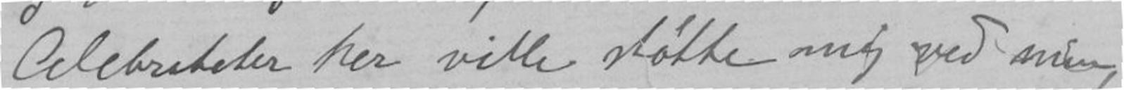Celebriteter her ville støtte mig ved min,
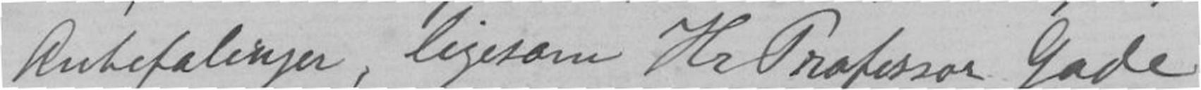Anbefalinger, ligesom Hr Professor Gade
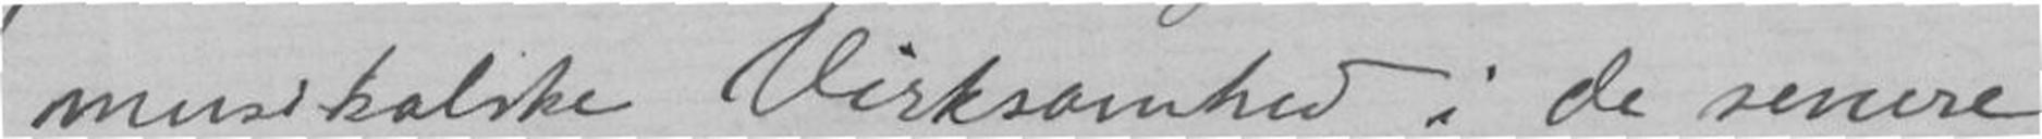musikaliske Virksomhed i de senere
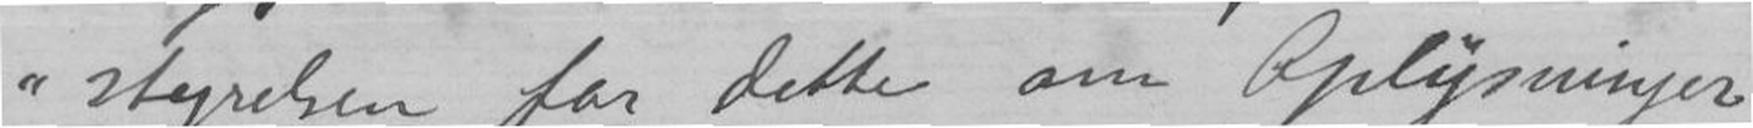=styrelsen for dette om Oplysninger
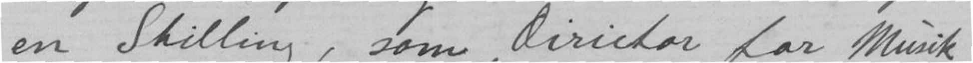en Stilling, som Dirictor for musik
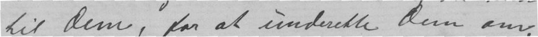til Dem, for at underette Dem om,
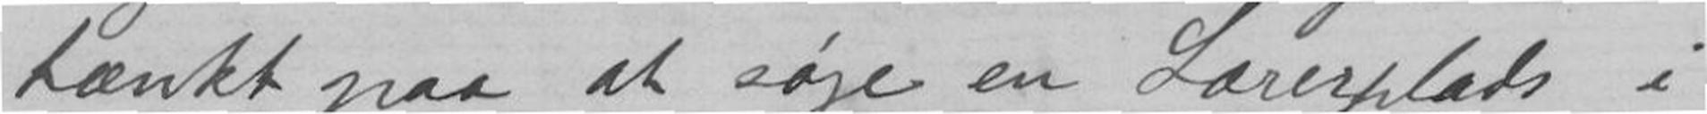tænkt paa at søge en Lærerplads i
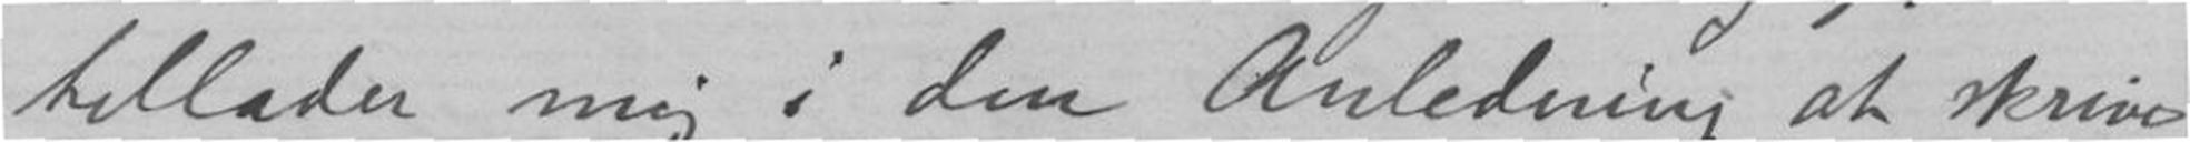tillader mig i den Anledning at skrive
